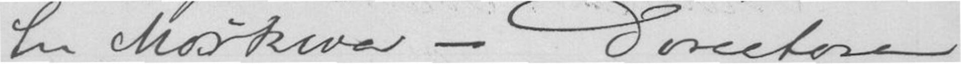til Moskwa - Derectoren
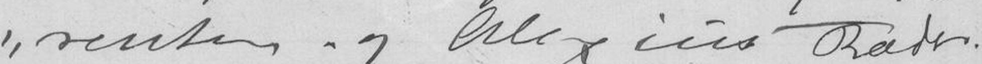renter og Alexius Ræder.
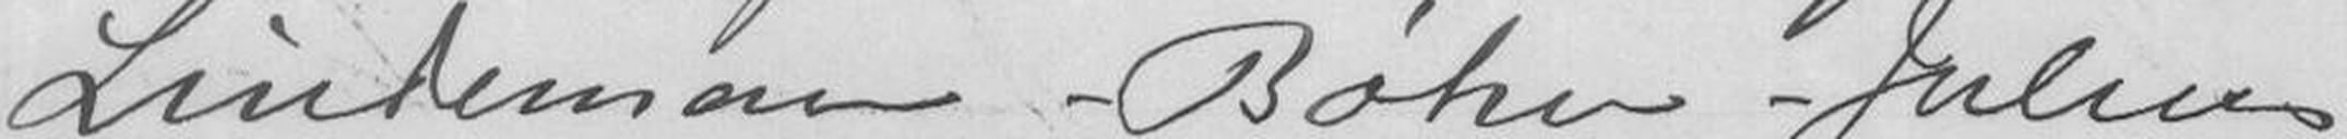Lindeman - Bøhn - Julius
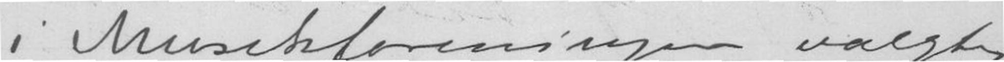i Musikforeningen valgte
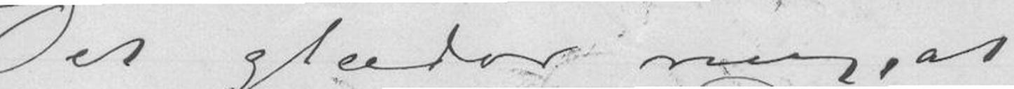Det glæder mig, at
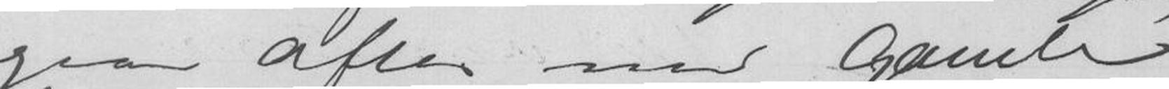igaar Aften med Gamle
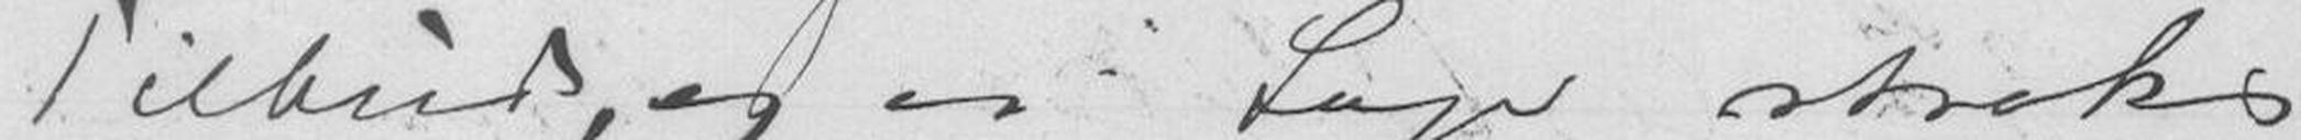Tilbud, og vi tager straks
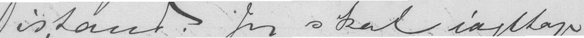istand. Jeg skal iagttage
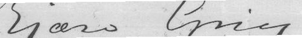Kjære Grieg
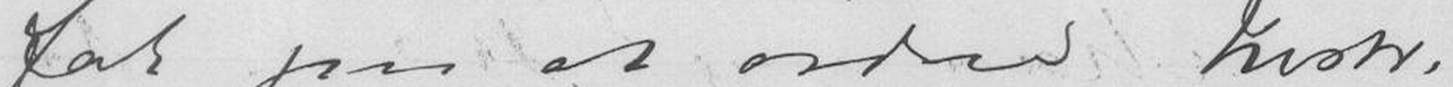fat paa at ordne Instr.
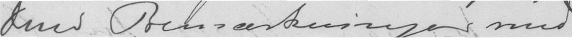Deres Bemærkninger med
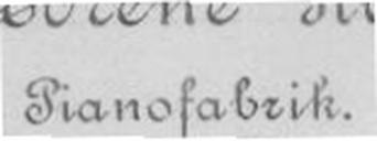Pianofabrik.
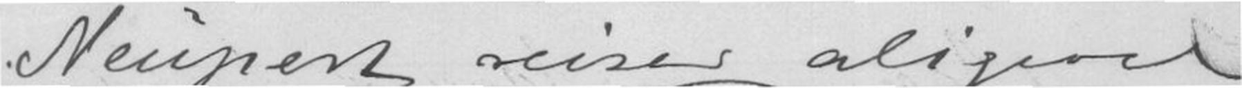Neupert reiser aligevel
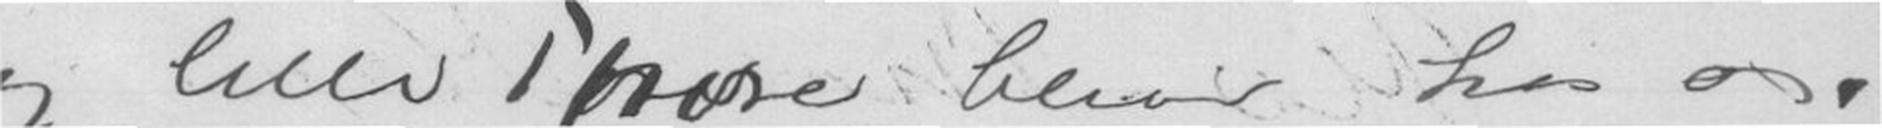og lille Thora bliver hos os.
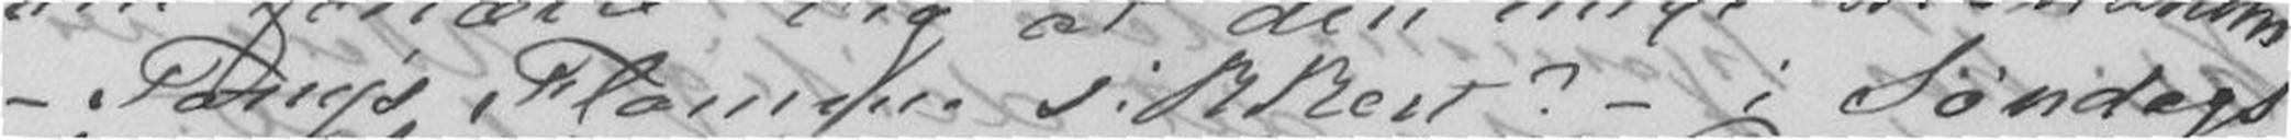- Tony's Flamme sikkert? - i Søndags
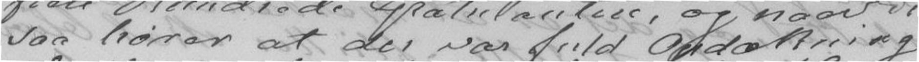saa hører at der var fuld Opdækning
